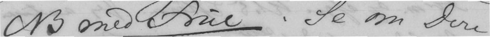NB med Frue. Se om dere
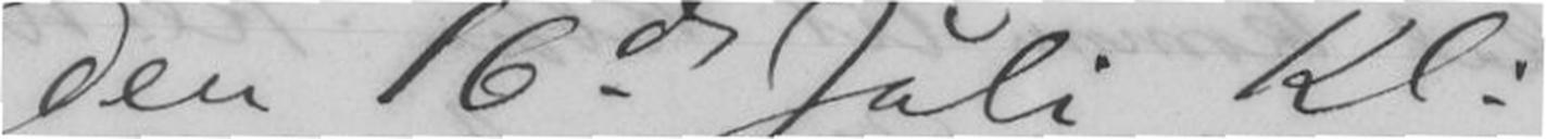den 16de Juli Kl.
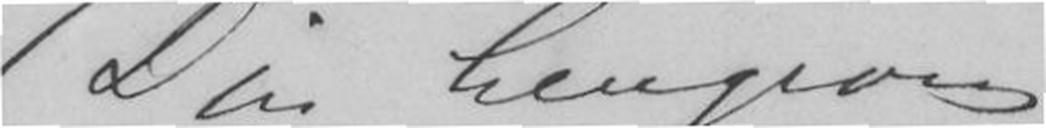Din hengivne
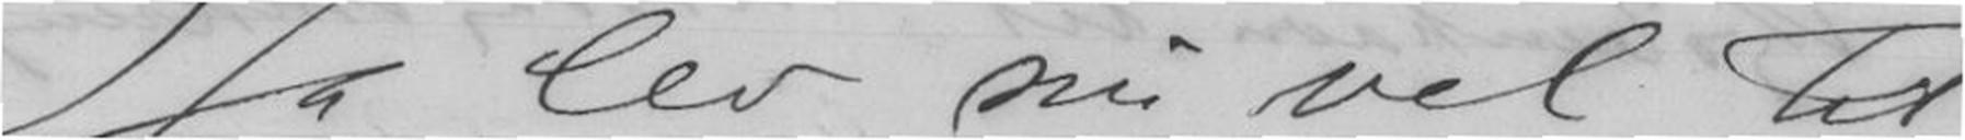Ja lev nu vel til
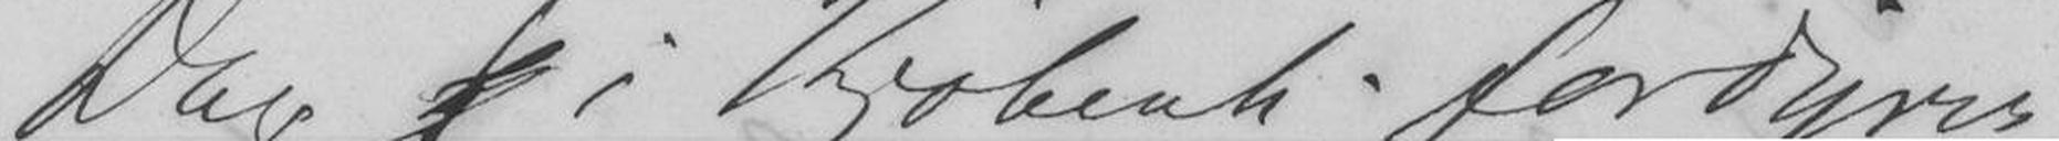Dage i Kjøbenh. fordyrer
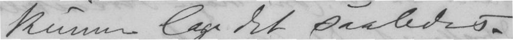kunne lage det saaledes.
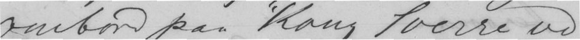ombord paa "Kong Sverre ved
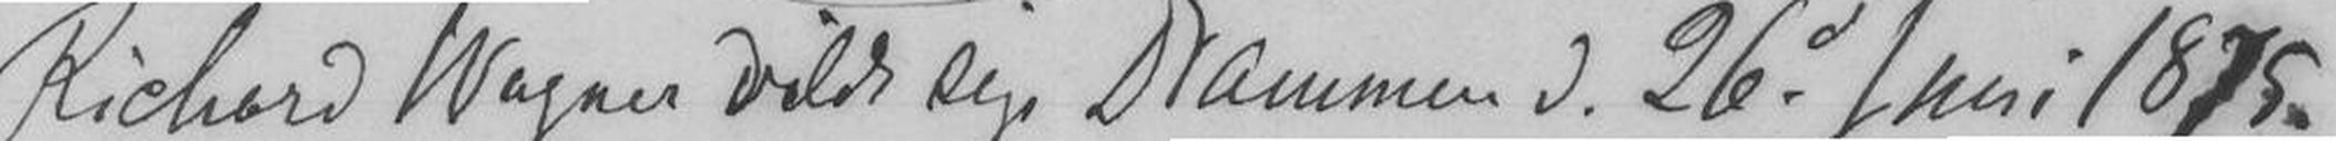Richard Wagner vilde sige Drammen d. 26d Juni 1875
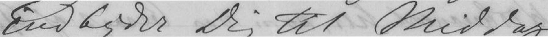indbyder Dig til Middag
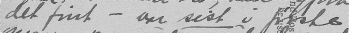det fint - var sist i første
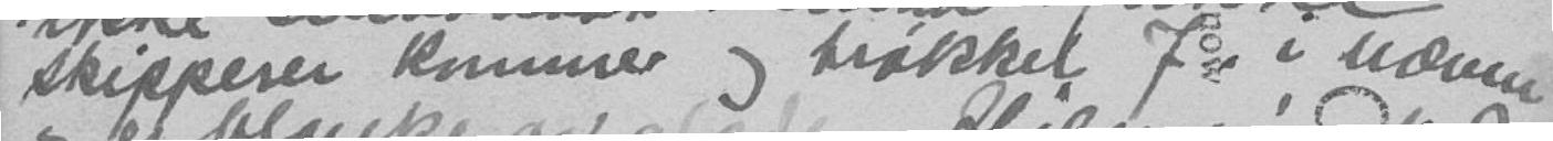skipperer kommer og trokkel J. i næven
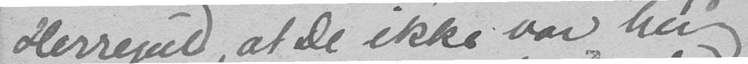Herregud, at De ikke var her og
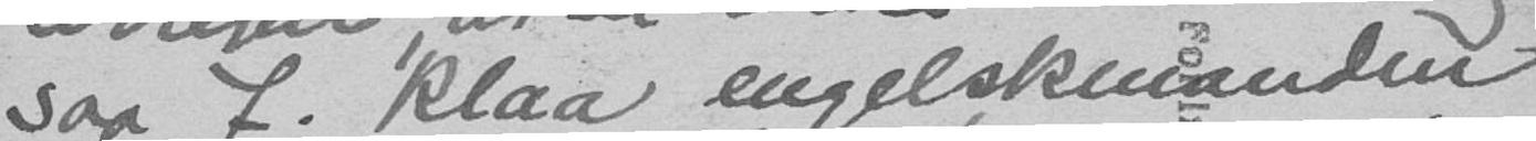saa J. klaa engelskmanden
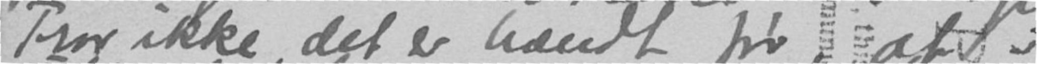Tror ikke det er hændt før at i
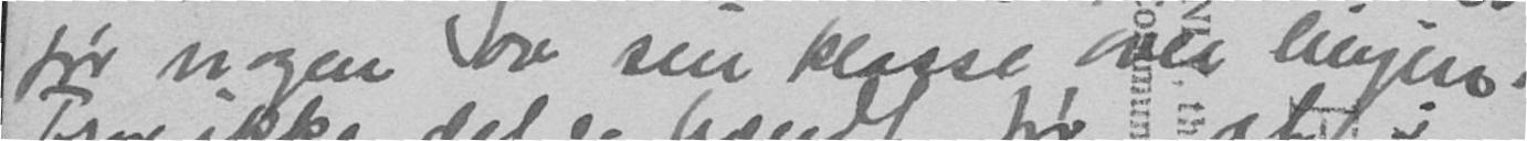før nogen av sin klasse over linjen.
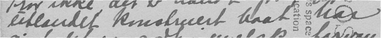utlandet konstruert baat har
